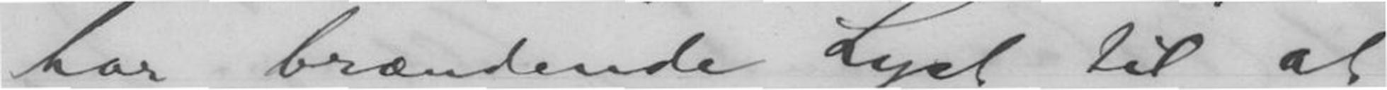har brændende Lyst til at
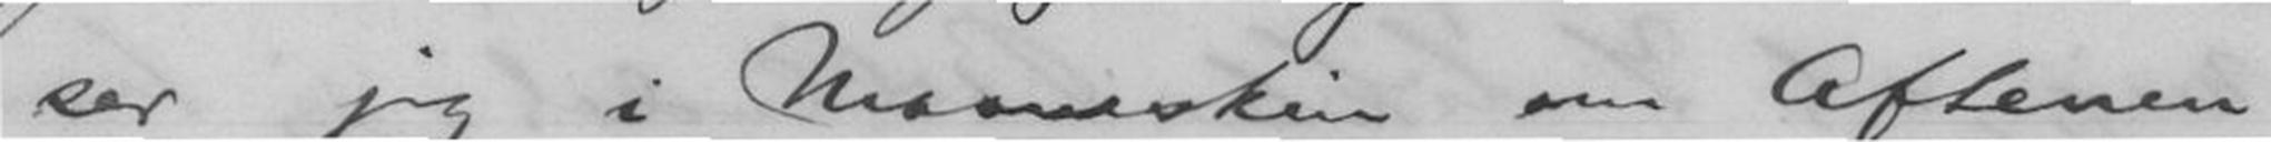ser jeg i Maaneskin om Aftenen
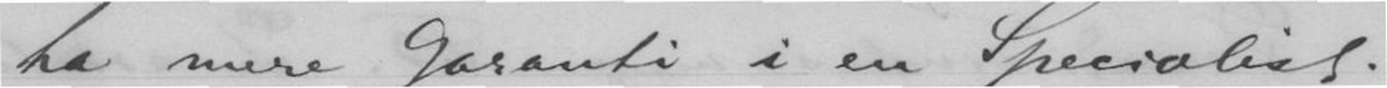ha mere Garanti i en Specialist.
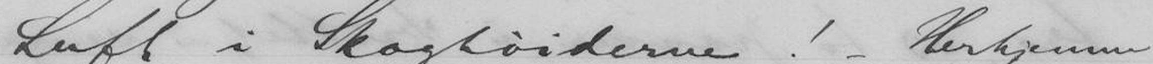Luft i Skoghøiderne! - Herhjemme
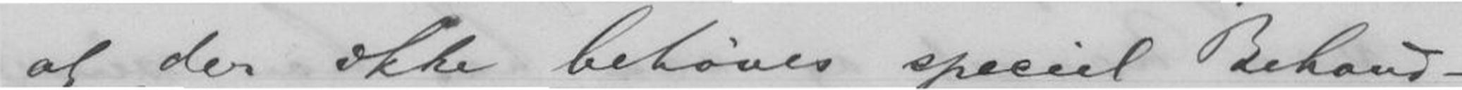at der ikke behøves spiciel Behand-
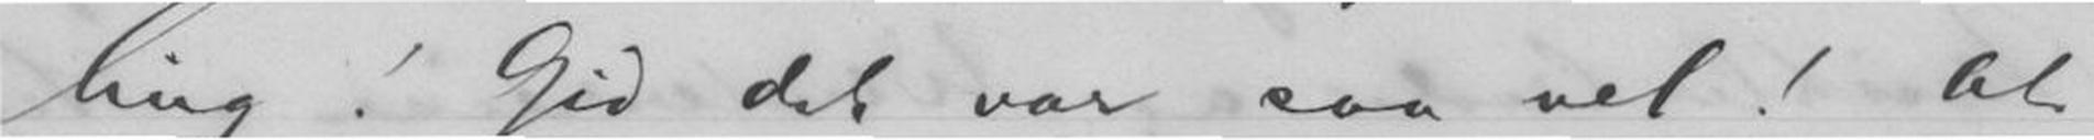ling! Gid det var saa vel! At
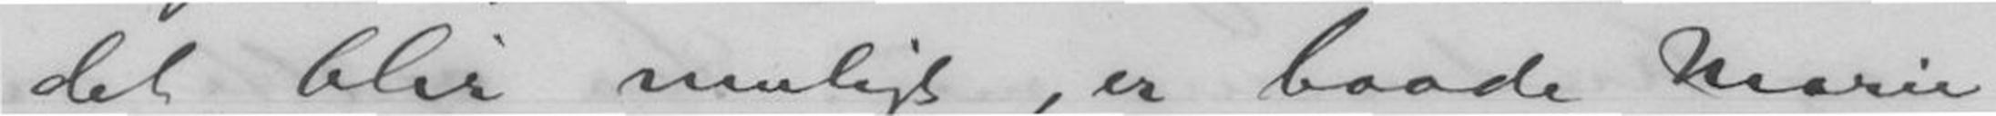det blir muligt, er baade Marie
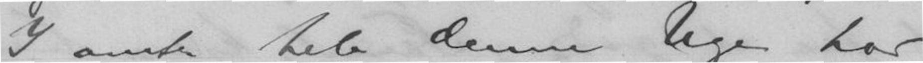I omtr hele denne Uge har
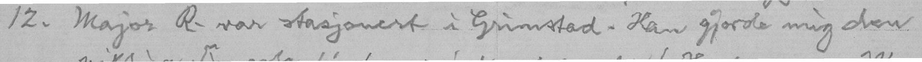12. Major R. var stasjonert i Grimstad. Han gjorde mig den
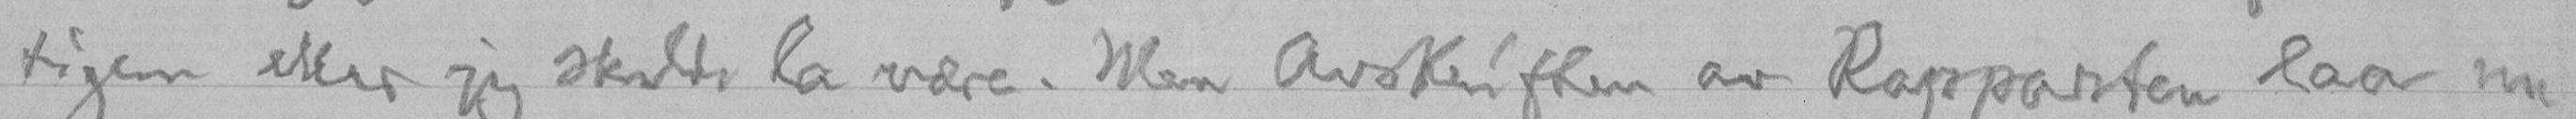tigen eller jeg skulde la være. Men Avskriften av Rapporten laa nu
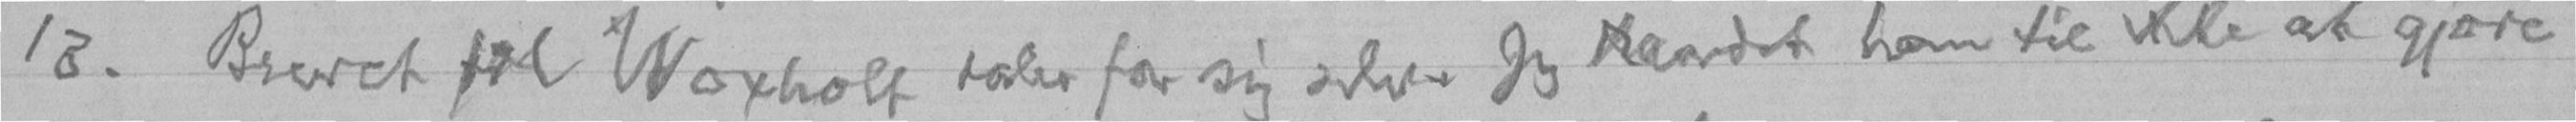13. Brevet til Woxholt taler for sig selv. Jeg raadet ham til ikke at gjøre
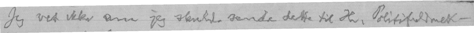Jeg vet ikke om jeg skulde sende dette til Hr. Politifuldmek-
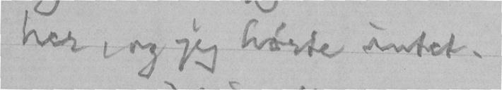her, og jeg hørte intet.
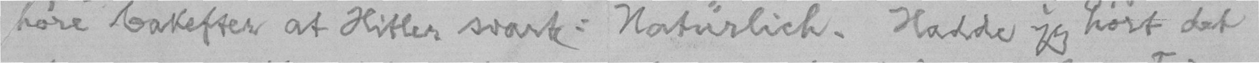høre bakefter at Hitler svarte: Natürlich. Hadde jeg hørt det
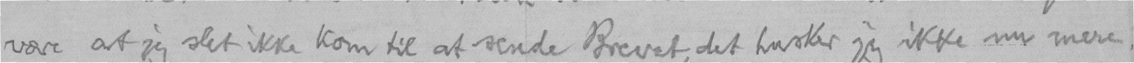være at jeg slet ikke kom til at sende Brevet, det husker jeg ikke nu mere.
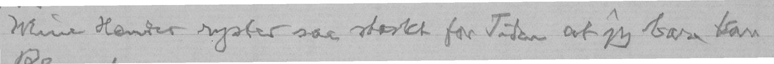Mine Hænder ryster saa stærkt for Tiden at jeg bare kan
